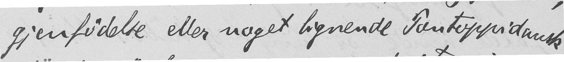gjenfødelse eller noget lignende Pontoppidansk
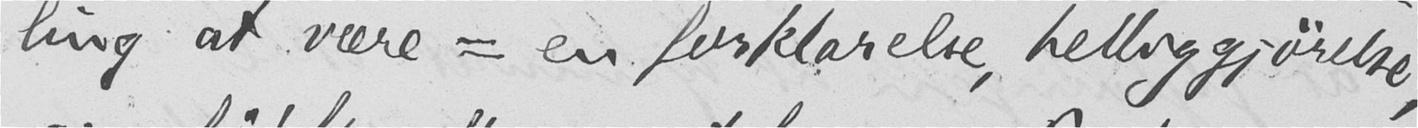ling at være = en forklarelse, helliggjørelse,
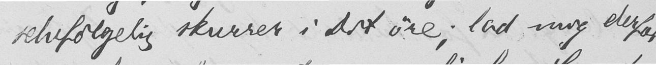selvfølgelig skurrer i Dit øre; lad mig derfor
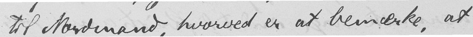til Nordmand, hvorved er at bemærke, at
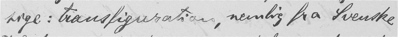sige: transfiguration, nemlig fra Svenske
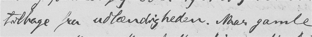tilbage fra udlændigheden. Naar gamle
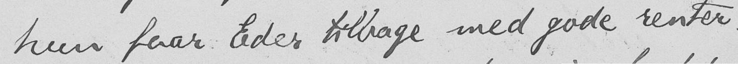hun faar Eder tilbage med gode renter.
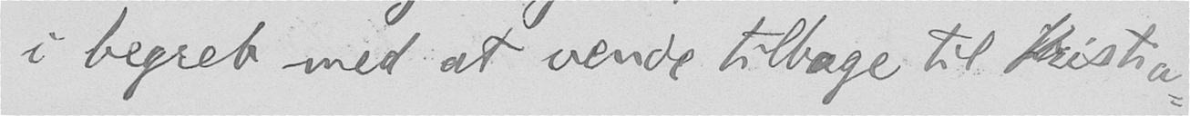i begreb med at vende tilbage til Kristia-
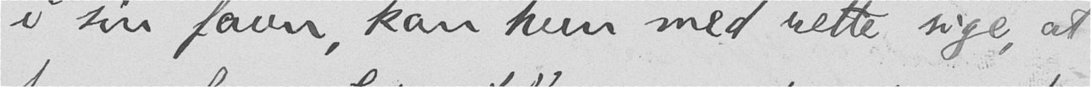i sin favn, kan hun med rette sige, at
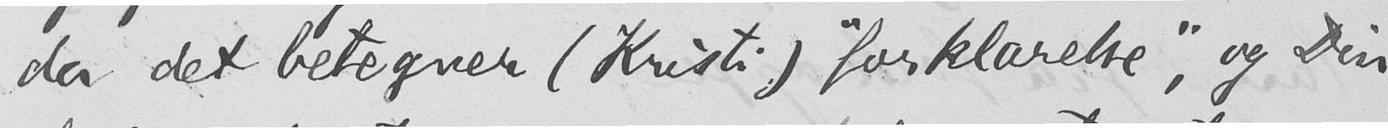da det betegner (Kristi) "forklarelse", og Din
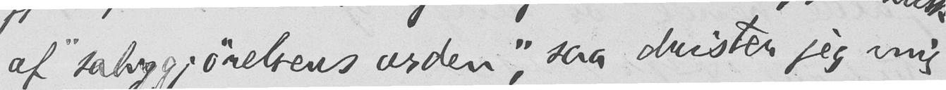af "saliggjørelsens orden", saa drister jeg mig
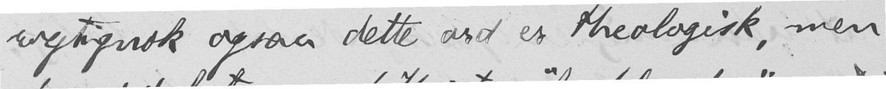rigtignok ogsaa dette ord er theologisk, men
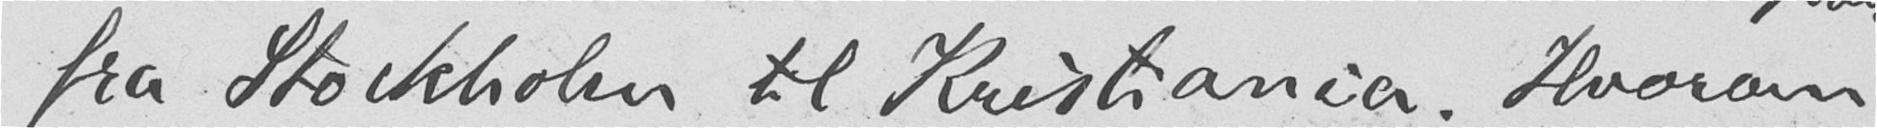fra Stockholm til Kristiania. Hvorom
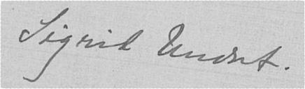Sigrid Undset.
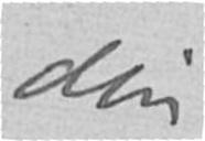din
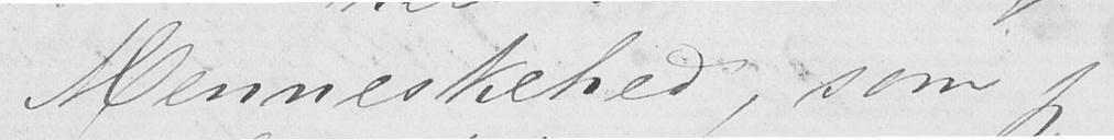Menneskehed, som jeg
